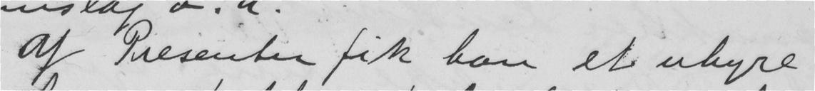Af Presenter fik han et uhyre
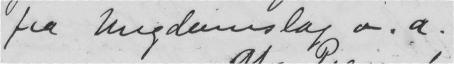fra Ungdomslag o.a.
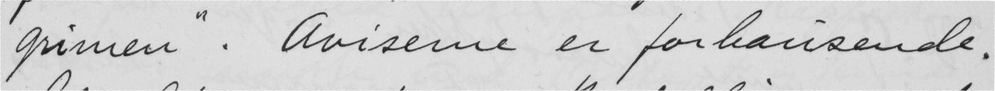grimen". Aviserne er forhausende.
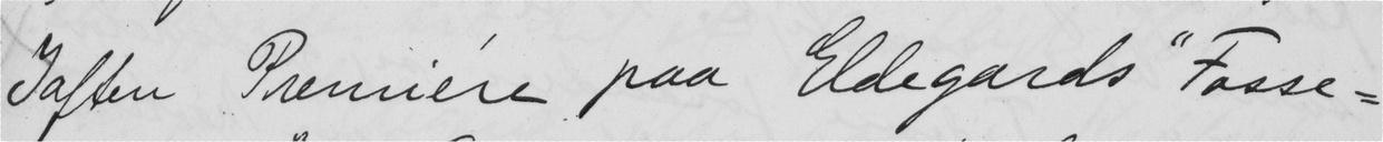Iaften Premiere paa Eldegards "Fosse-
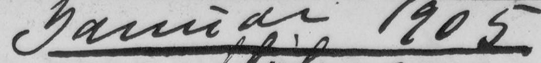Januar 1905
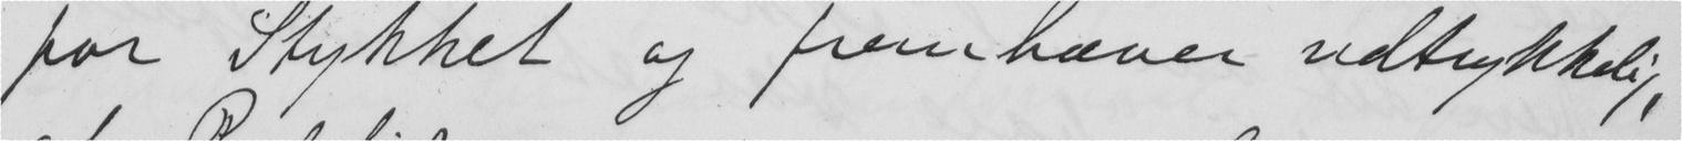for Stykket og fremhæver udtrykkelig,
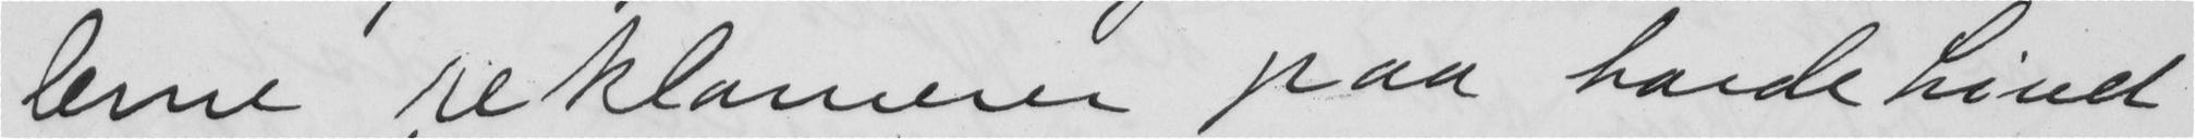lerne reklamerer paa harde Livet
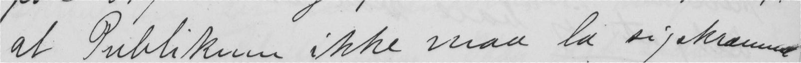at Publikum ikke maa la sig skræmme
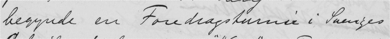begynde en Foredragsturne i Sveriges
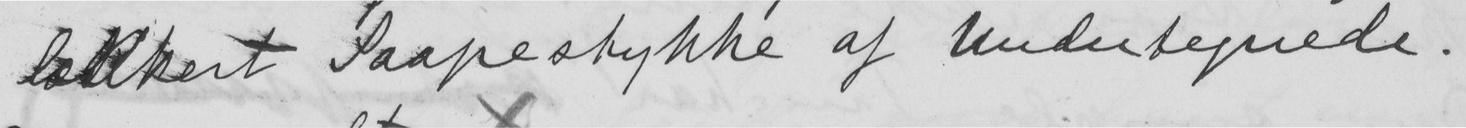lækkert Saapestykke af Undertegnede.
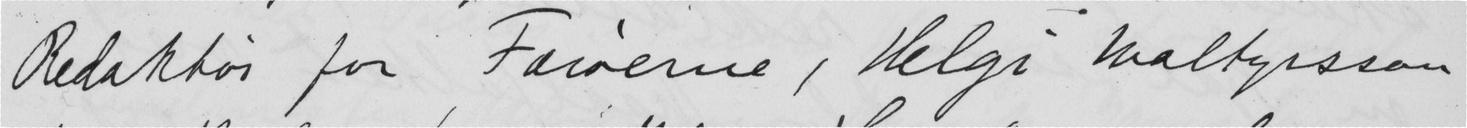Redaktør for Færøerne, Helgi Waltyrsson
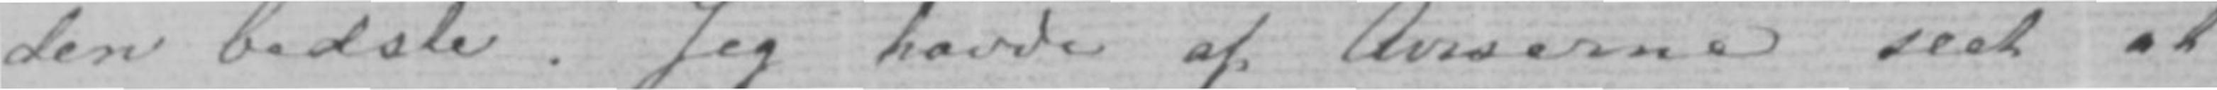den bedste. Jeg havde af Aviserne seet at
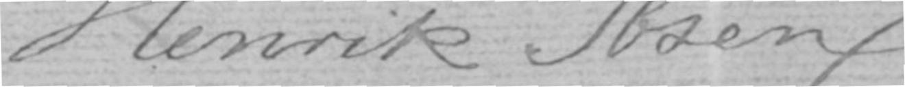Henrik Ibsen.
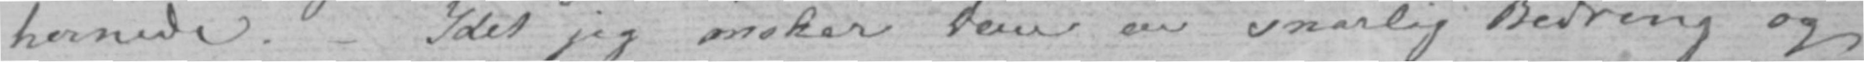hernede.- Idet jeg ønsker Dem en snarlig Bedring og
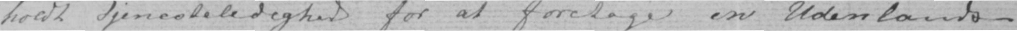holdt Tjenesteledighed for at foretage en Udenlands-
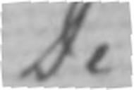De
